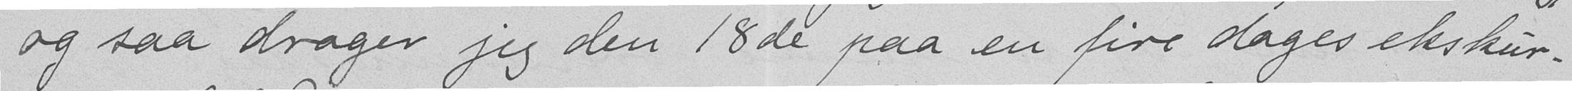og saa drager jeg den 18de paa en fire dages ekskur-
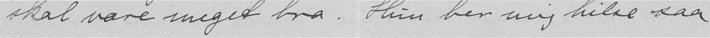skal være meget bra. Hun ber mig hilse saa
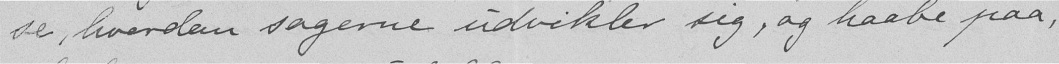se, hvordan sagerne udvikler sig, og haabe paa,
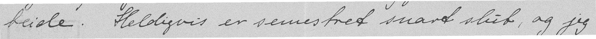beide. Heldigvis er semestret snart slut, og jeg
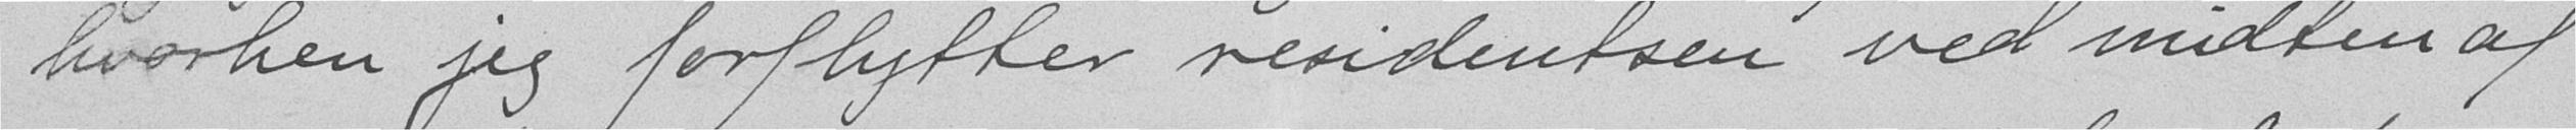hvorhen jeg forflytter residentsen ved midten af
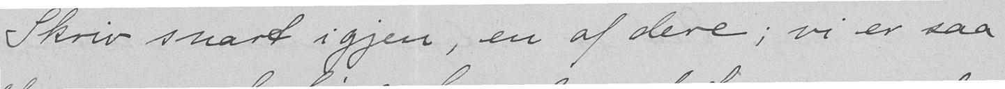Skriv snart igjen, en af dere; vi er saa
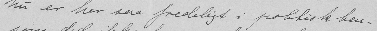Nu er her saa fredeligt i politisk hen-
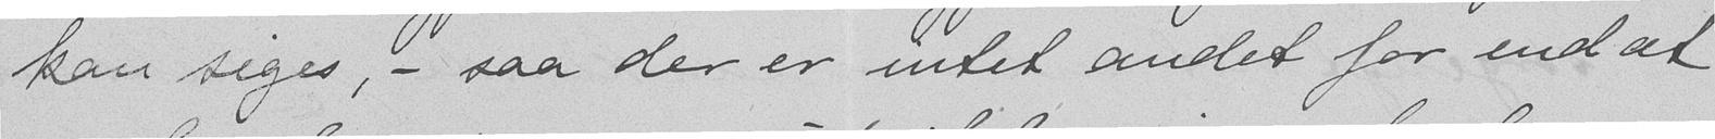kan siges, - saa der er intet andet for end at
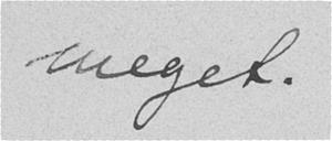meget.
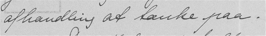afhandling at tænke paa.
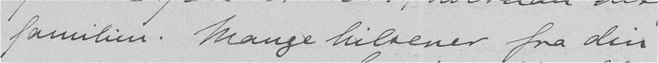familien. Mange hilsener fra din
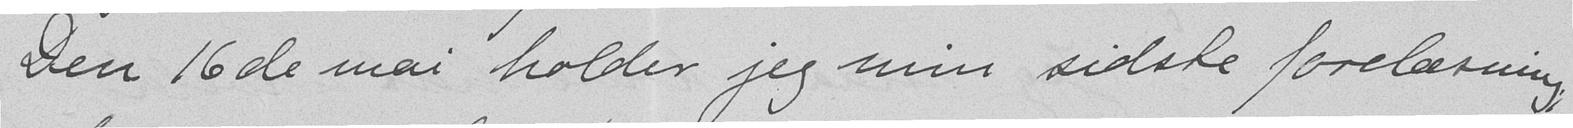Den 16de mai holder jeg min sidste forelæsning;
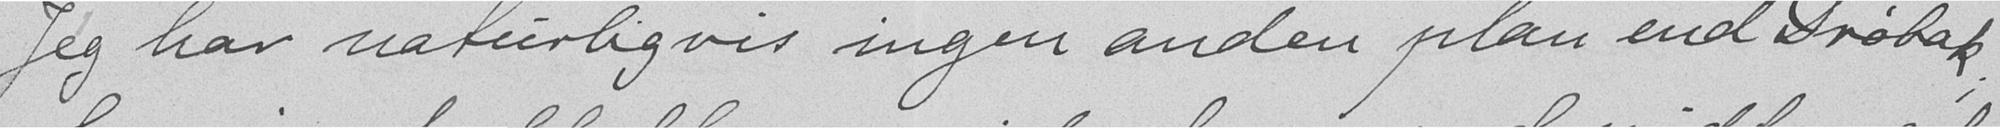Jeg har naturligvis ingen anden plan end Drøbak;
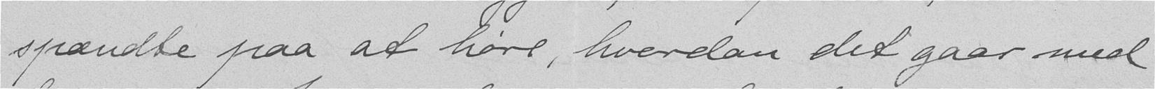spændte paa at høre, hvordan det gaar med
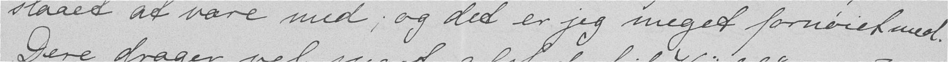slaaet at være med; og det er jeg meget fornøiet med.
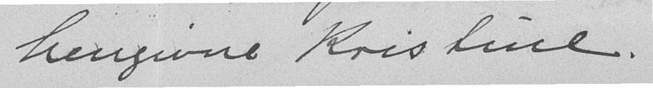hengivne Kristine.
